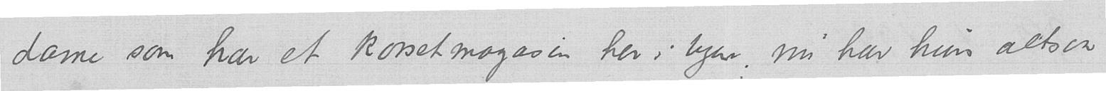dame som har et korsetmagasin her i byen, nu har hun altsaa
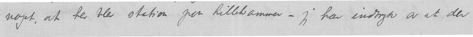noget, at her blev station paa Lillehammer – jeg har indtryk av at der
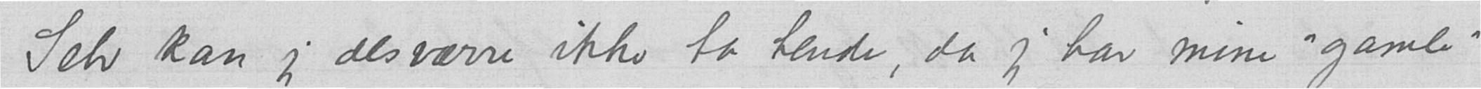Selv kan jeg desværre ikke ta hende, da jeg har mine "gamle"
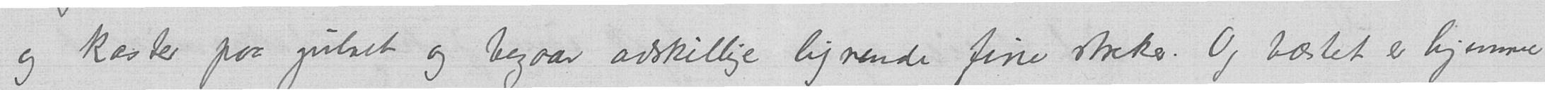og kaster paa gulvet og begaar adskillige lignende fine streker. Og bæstet er hjemme
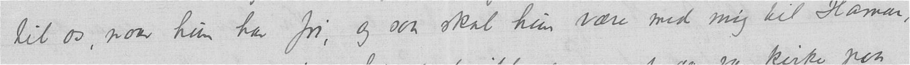til os, naar hun har fri, og saa skal hun være med mig til Hamar,
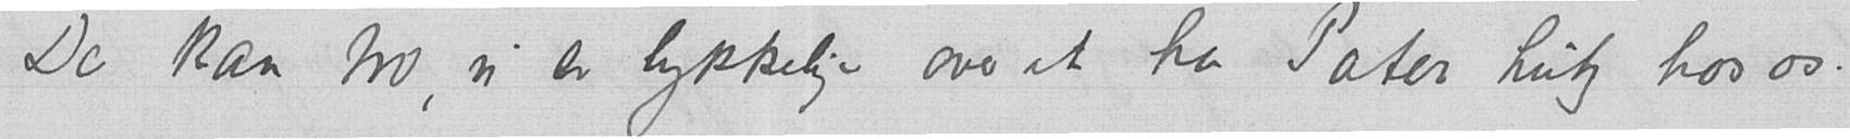De kan tro, vi er lykkelige over at ha Pater Lutz hos os.
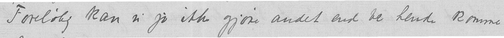Foreløbig kan vi jo ikke gjøre andet end be hende komme
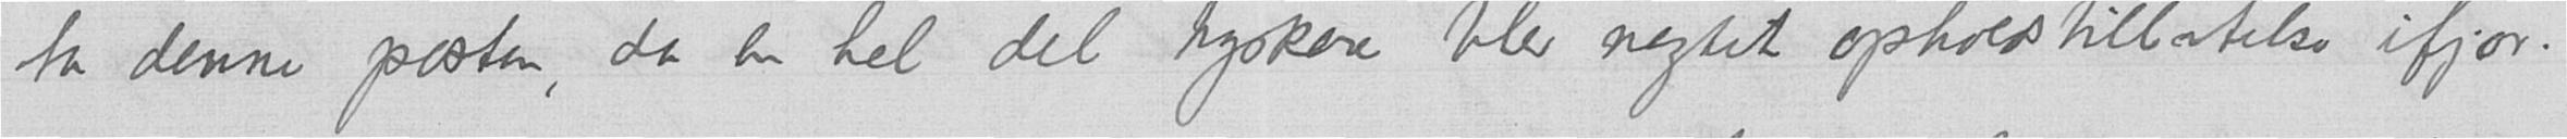ta denne posten, da en hel del tyskere blev negtet opholdstillatelse ifjor.
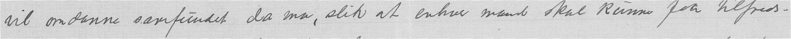vil omdanne samfundet, da ma, slik at enhver mand skal kunne faa tilfreds-
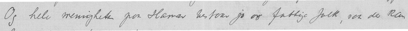Og hele menigheten paa Hamar bestaar jo av fattige folk, saa der kan
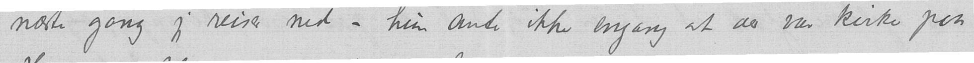næste gang jeg reiser ned – hun ante ikke engang at der var kirke paa
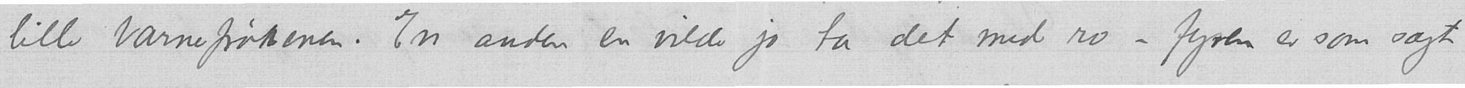lille barnefrøkenen. En anden en vilde jo ta det med ro – fyren er som sagt
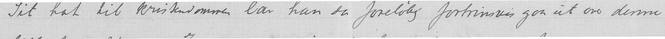Sit hat til kristendommen lar han da foreløbig fortrinsvis gaa ut over denne
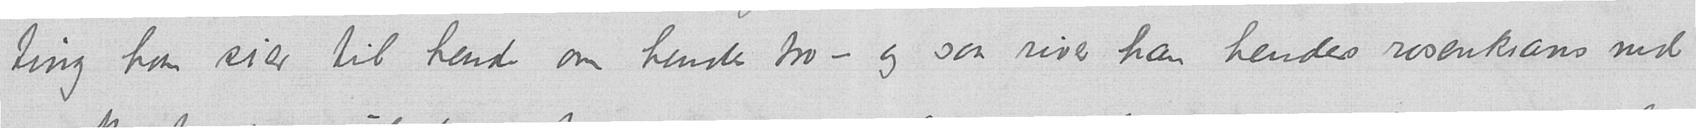ting han sier til hende om hendes tro – og saa river han hendes rosenkrans ned
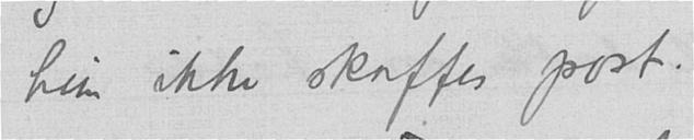hun ikke skaffes post.
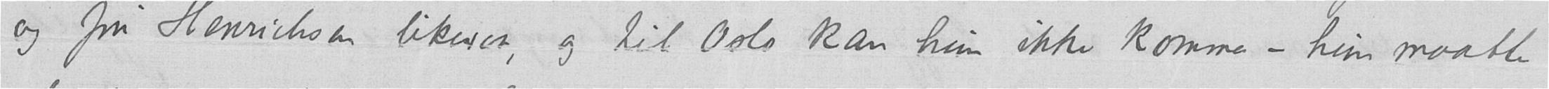og fru Henrichsen likesaa, og til Oslo kan hun ikke komme – hun maatte
